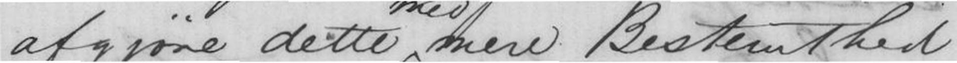afgjøre dette med mere Bestemthed
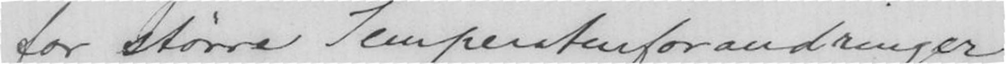for større Temperaturforandringer
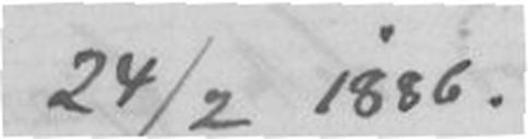24/2 1886.
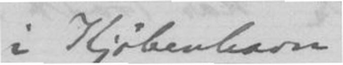i Kjøbenhavn
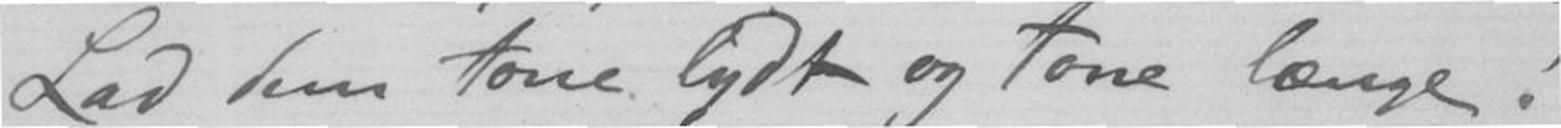Lad dem tone lydt og tone længe!
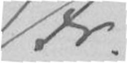J Fr
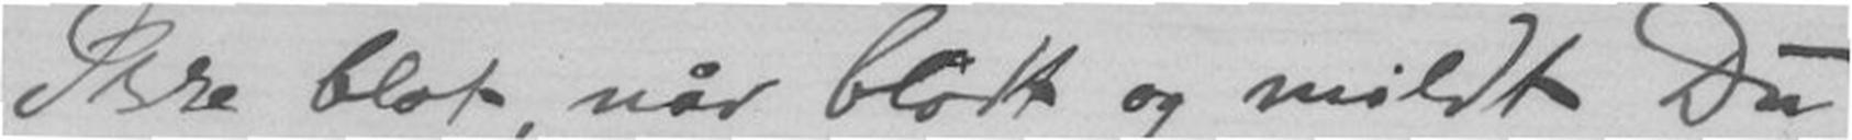Ikke blot, når blott og mildt Du
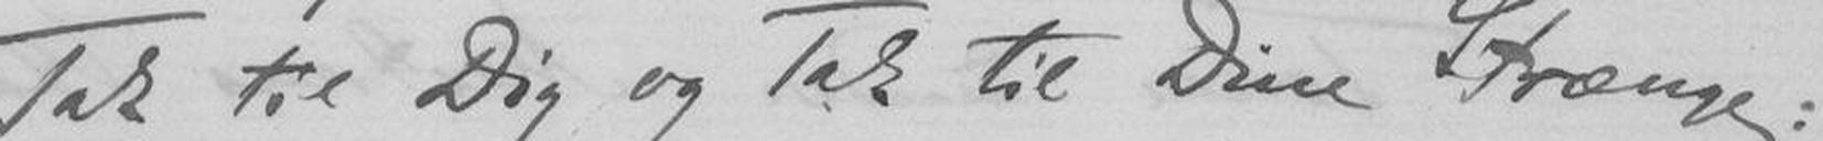Tak til Dig og Tak til Dine Strænge:
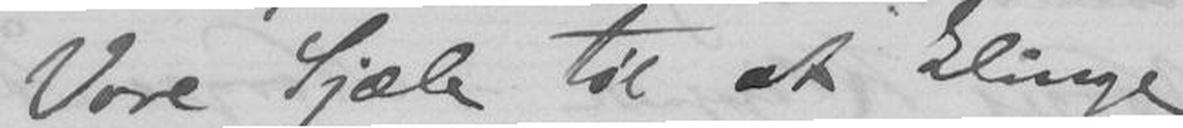Vore Sjæle til at klinge
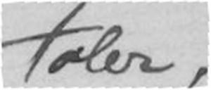taler,
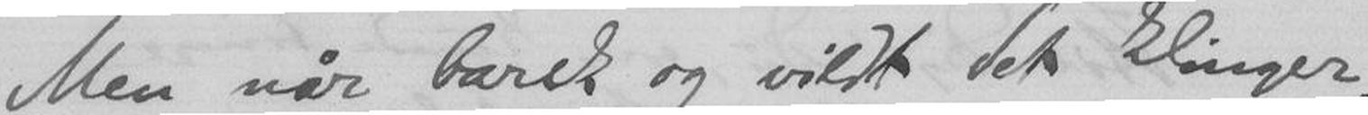Men når barsk og vildt det klinger,
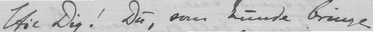Hil Dig! Du, som kunde bruge
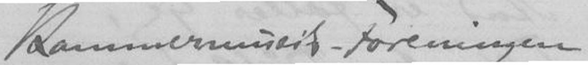Kammermusik-Foreningen
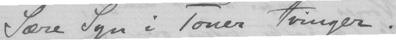Sære Syn i Toner tvinger.
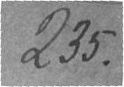235.
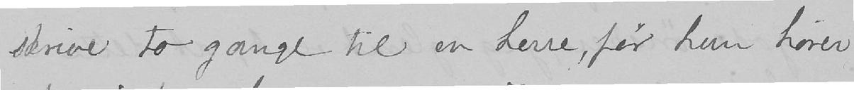skrive to gange til en herre, før hun hører
